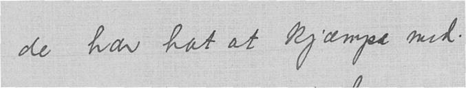de har hat at kjæmpe med.
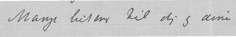Mange hilsener til dig og dine
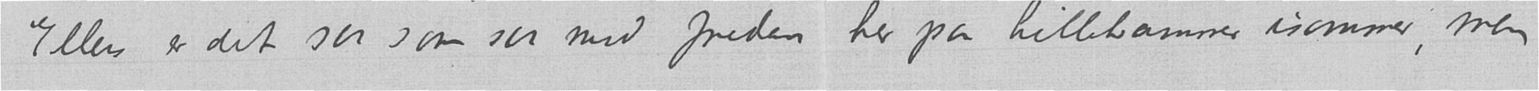Ellers er det saa som saa med freden her paa Lillehammer isommer, men
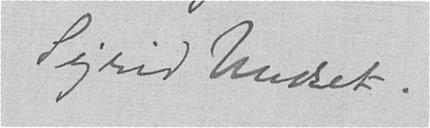Sigrid Undset.
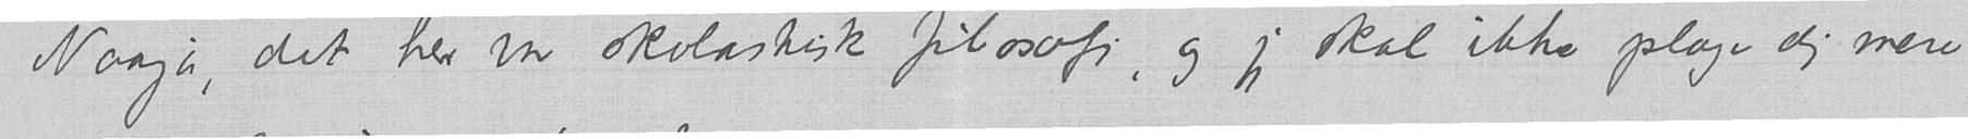Naaja, det her var skolastisk filosofi, og jeg skal ikke plage dig mere
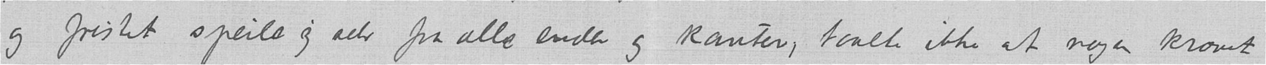og fristet speile sig selv fra alle ender og kanter, taalte ikke at nogen krævet
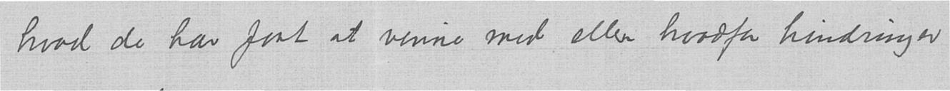hvad de har faat at vinne med, eller hvadfor hindringer
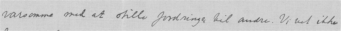varsomme med at stille fordringer til andre. Vi vet ikke
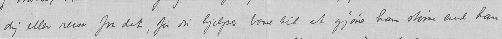dig eller reise fra det, for du hjelper bare til at gjøre ham større end han
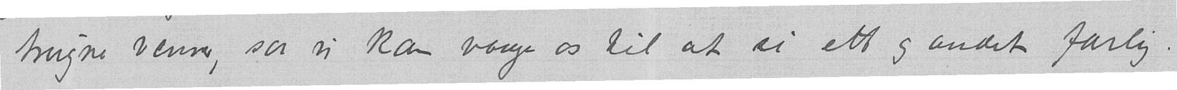trugne venner, saa vi kan vaage os til at si ett og andet farlig.
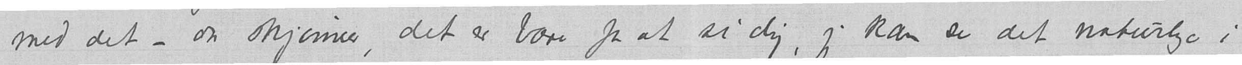med det – du skjønner, det er bare for at si dig, jeg kan se det naturlige i
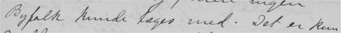Byfolk kunde tages med. Det er kun
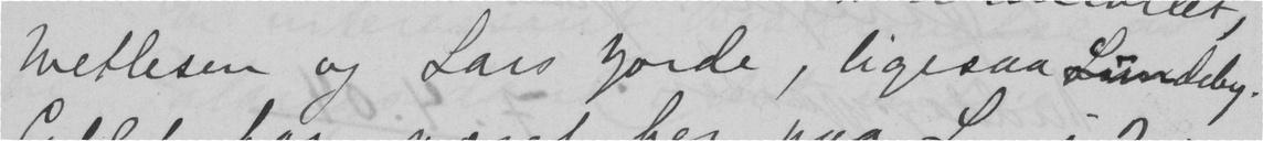Wetlesen og Lars Jorde, ligesaa Lundeby.
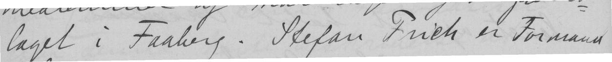laget i Faaberg. Stefau Frich er Formand
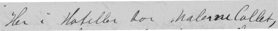Her i Hoteller bor malerme Collet,
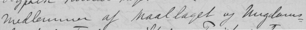medlemmer af Maallaget og Ungdoms-
